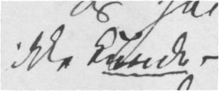ikke kunde -
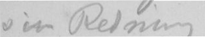sin Redning.
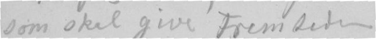som skal give Fremtiden
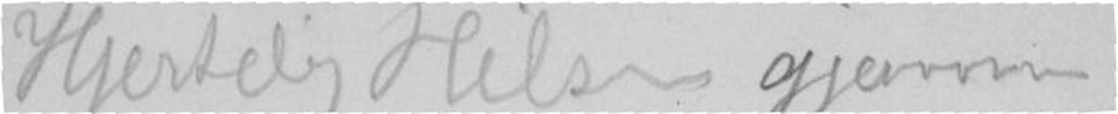Hjertelig Hilsen gjennem
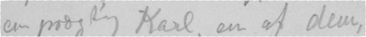en prægtig Karl, en af dem,
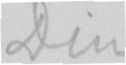Din
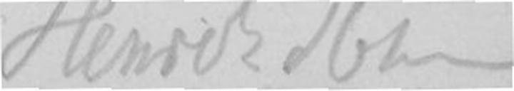Henrik Ibsen.
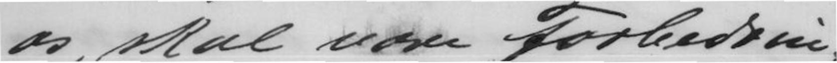os, skal være Forbedrin-
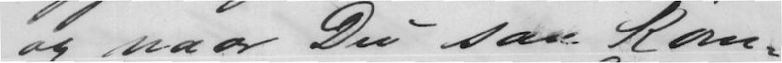Og naar Du saa kom-
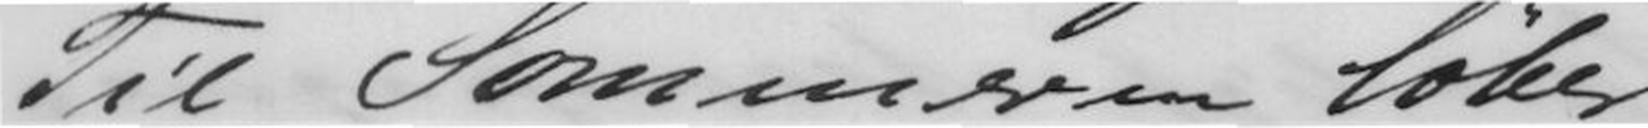Til Sommeren løber
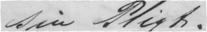sin Pligt.
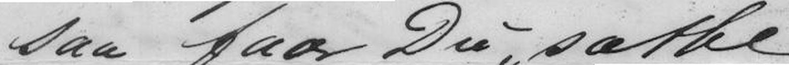saa faar Du sætte
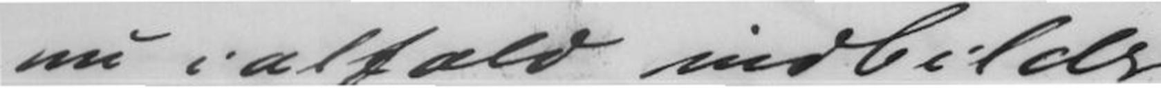nu ialfald indbilder
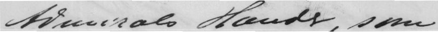Admirals Hænder, som
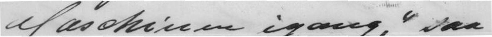Maschinen igang, saa
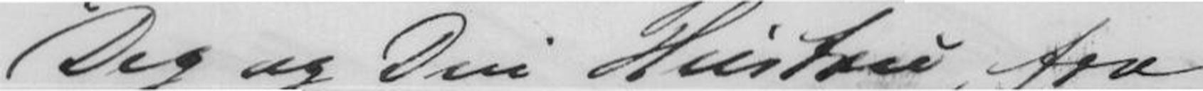Dig og Din Hustru, fra
